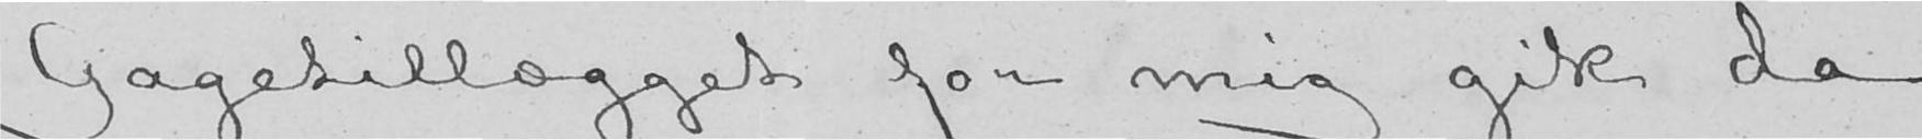Gagetillægget for mig gik da
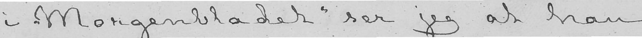i «Morgenbladet» ser jeg at han
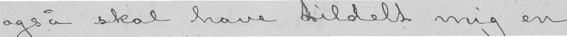også skal have tildelt mig en
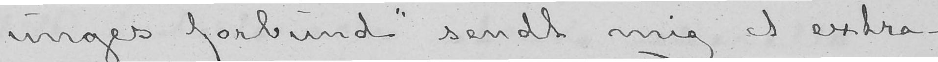unges forbund» sendt mig et extra-
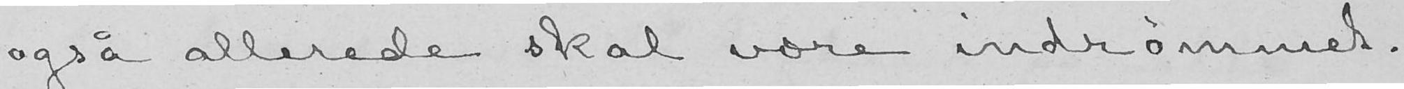også allerede skal være indrømmet.
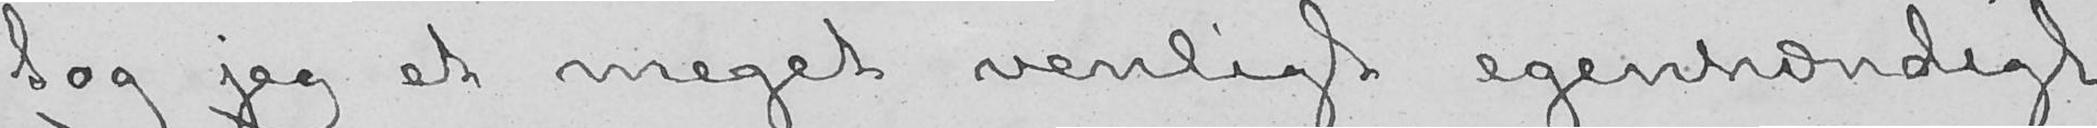tog jeg et meget venligt egenhændigt
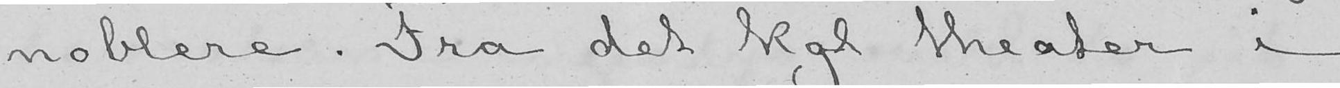noblere. Fra det kgl theater i
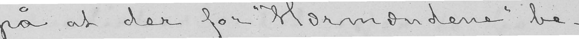på at der for «Hærmændene» be-
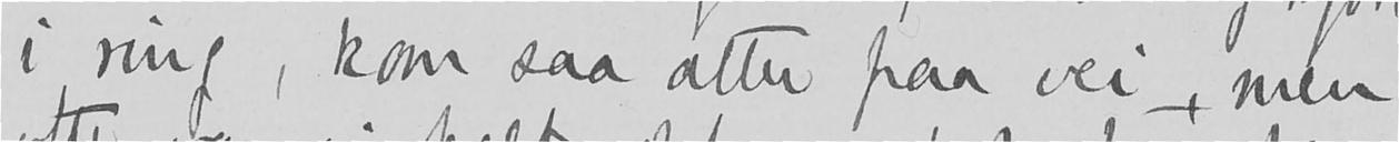i ring, kom saa atter paa vei, men
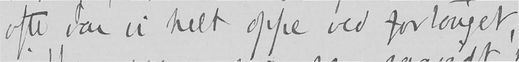ofte var vi helt oppe ved fortouget,
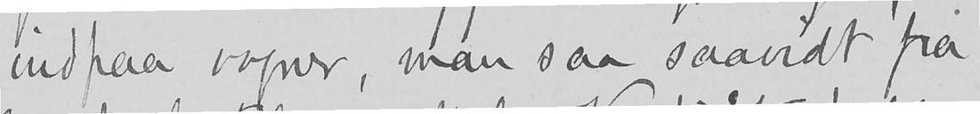indpaa vogner, man saa saavidt fra
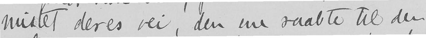mistet deres vei, den ene raabte til den
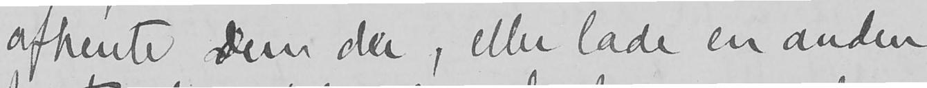afhente Dem der, eller lade en anden
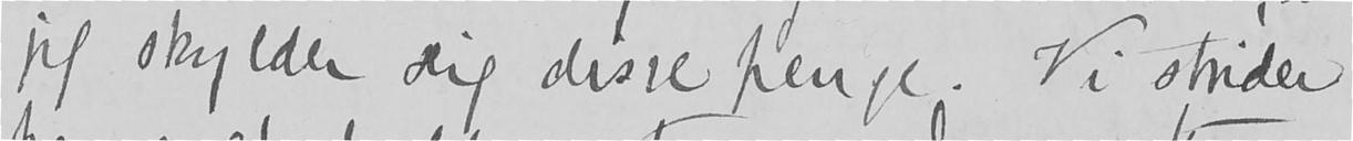jeg skylder dig disse penge. Vi strider
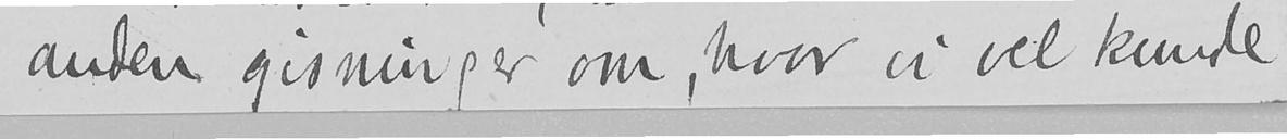anden gisninger om, hvor vi vel kunde
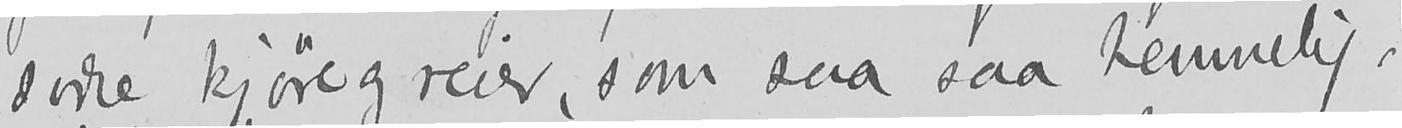svære kjøregreier som saa saa hemmelig-
